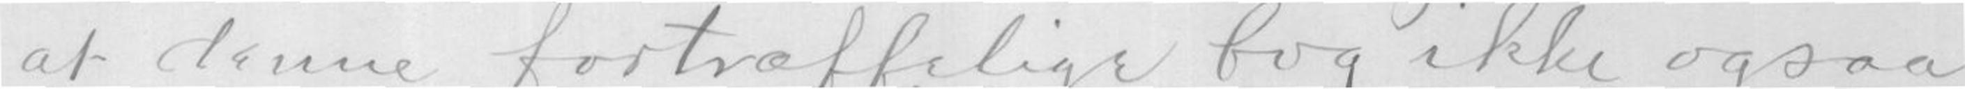at denne fortræffelige bog ikke ogsaa
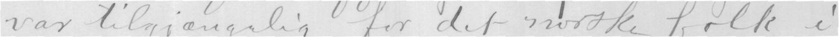var tilgjængelig for det norske folk i
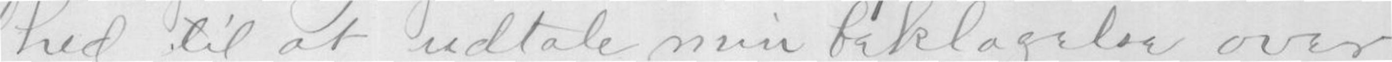hed til at udtale min beklagelse over,
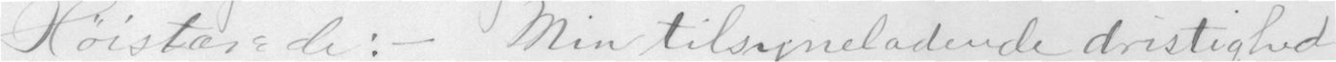Høistærede: - Min tilsyneladende dristighed
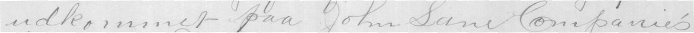udkommet paa John Sande Companie's
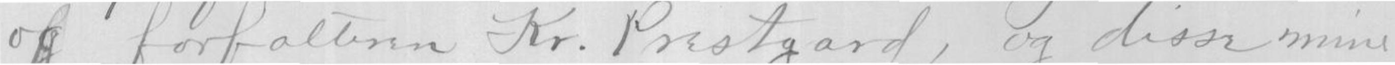og forfatteren Kr.Prestgard, og disse mine
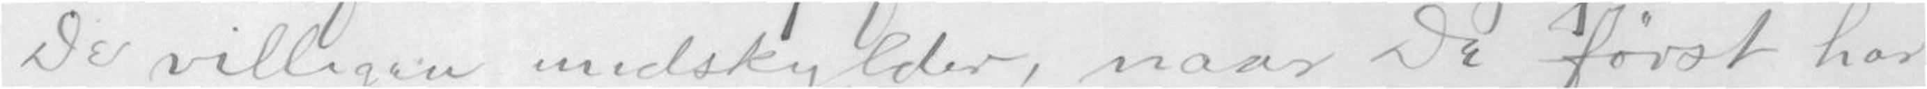De villigen medskylder, naar De først har
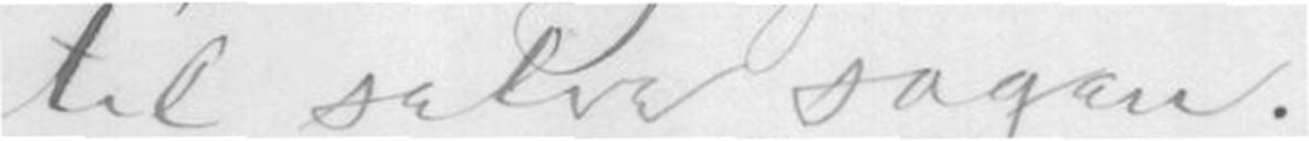til selve sagan.
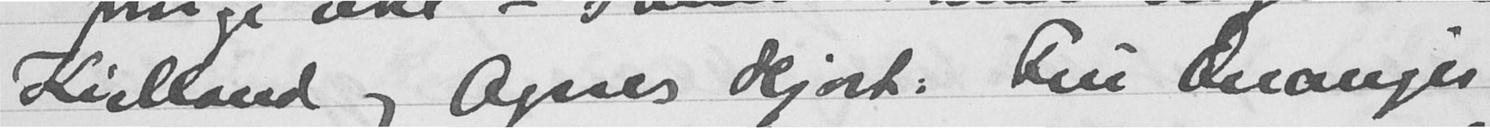Kielland og Agnes Hjort. Fru Quanjer
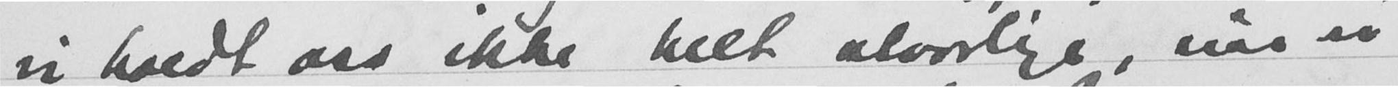vi holdt oss ikke helt alvorlige, når vi
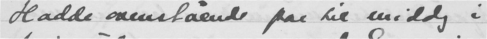Hadde overstående par til middag i
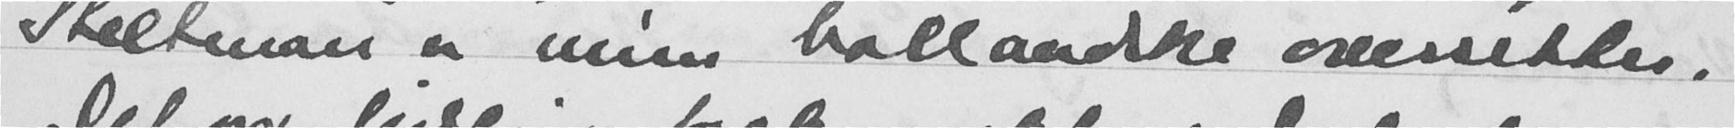Steltman er min hollandske oversetter.
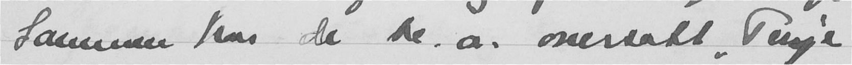Sammen har de bl. a. oversatt "Vinje
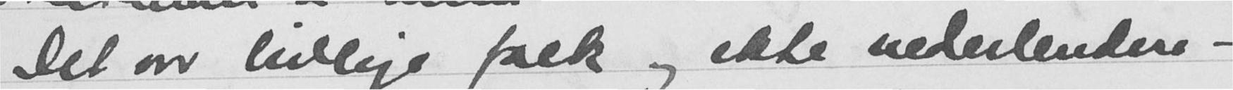Det var livlige folk og ekte nederlendere -
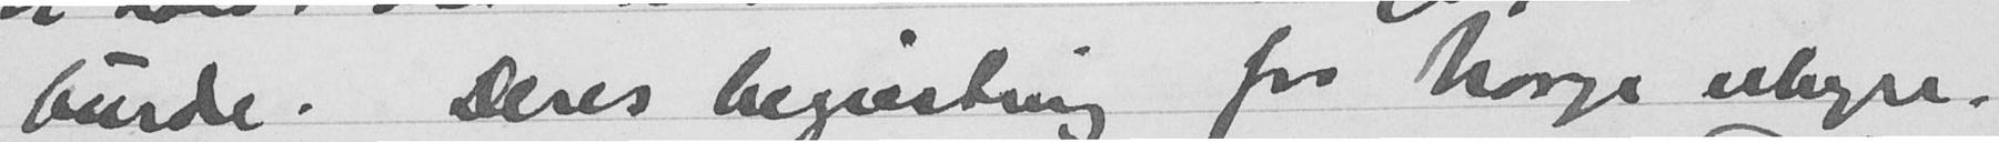burde. Deres begeistring for Norge uhyre.
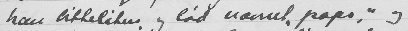han bitteliten og lød navnet "paps," og
